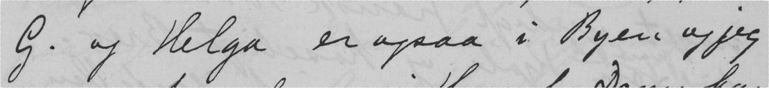G. og Helga er ogsaa i Byen og jeg
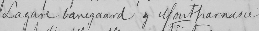Lagare banegaard og Montparnasse
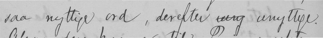saa nyttige ord, derefter mig unyttige.
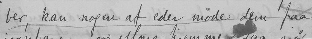ber, kan nogen af eder møde dem paa
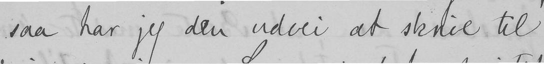saa har jeg den udvei at skrive til
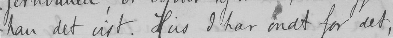han det vist. Hvis I har ondt for det,
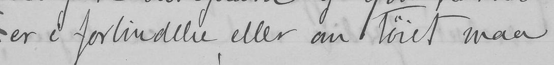er i forbindelse eller om tøiet maa
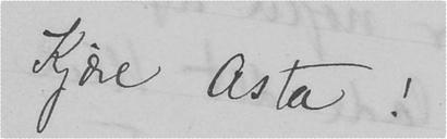Kjære Asta!
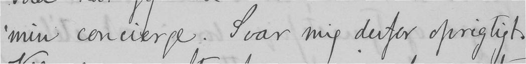min concierge. Svar mig derfor oprigtigt.
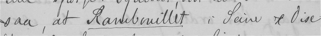saa, at Rambouillet i Seine et Oise
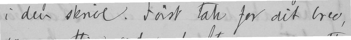i den skrive. Først tak for dit brev,
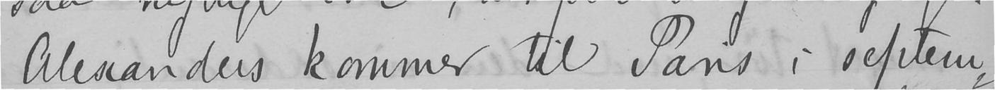Alexanders kommer til Paris i septem-
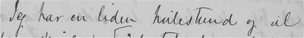Jeg har en liden hvilestund og vil
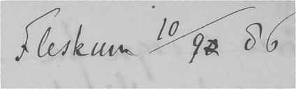Fleskum 10/9 86.
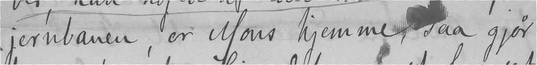jernbanen, er Mons hjemme, saa gjør
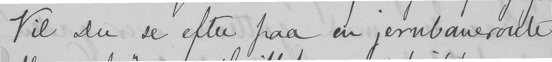Vil Du se efter paa en jernbaneroute
